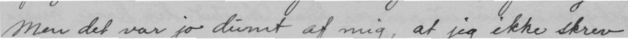Men det var jo dumt af mig, at jeg ikke skrev
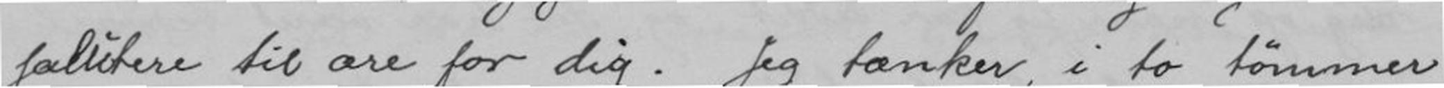salutere til ære for dig. Jeg tænker i to tømmer
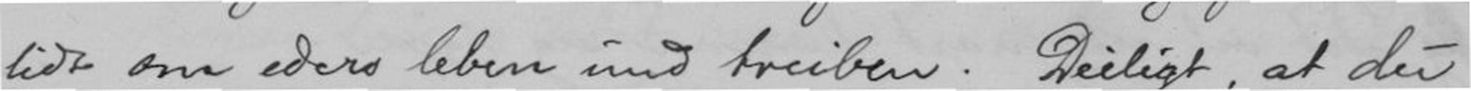lidt om eders leben und treiben. Deiligt, at du
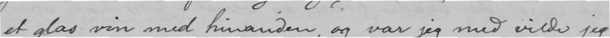et glas vin med hindanden, og var jeg med vilde jeg
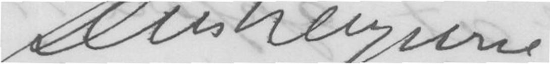Din hengivne
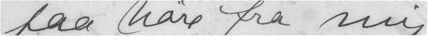faa høre fra mig
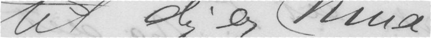til dig og Nina
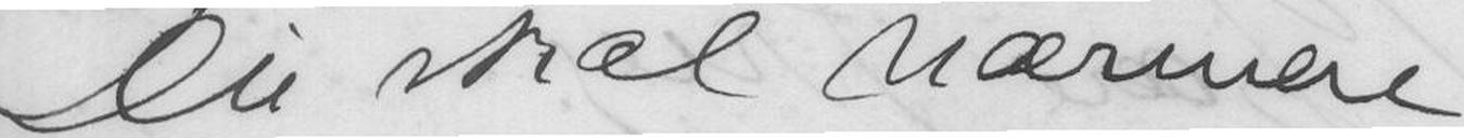Du skal nærmere
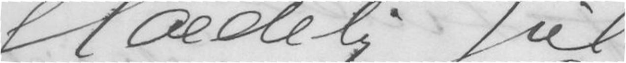glædelig jul
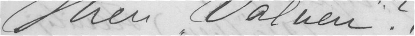Men Völven?!
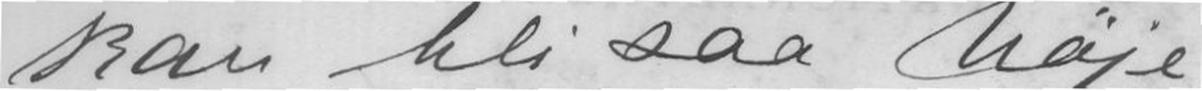kan bli saa høje
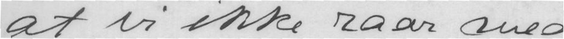at vi ikke raar med
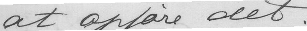at opføre det.
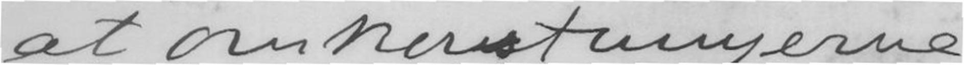at omkostningerne
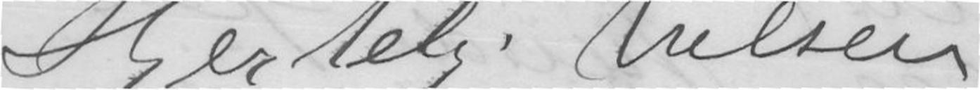Hjertelig hilsen
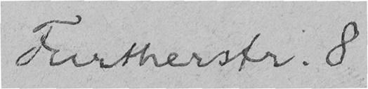Furtherstr. 8
